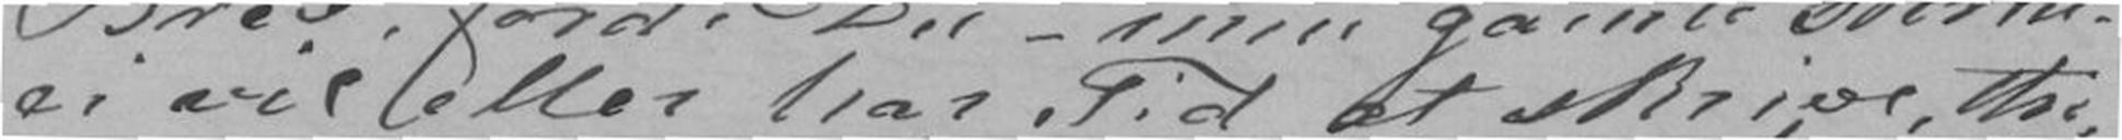ei vil eller har Tid at skrive, thi
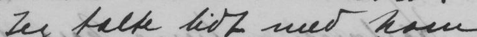Jeg talte lidt med ham
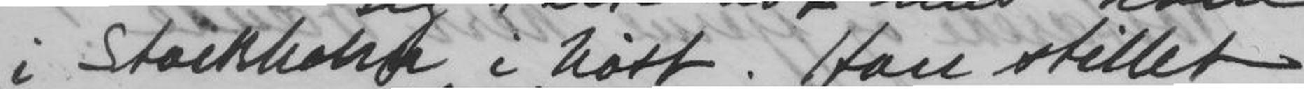i Stockholm i høst. Han strillet
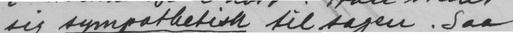sig sympathetisk til sagen. Saa
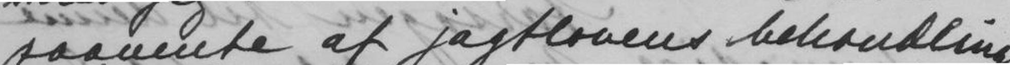paavente af jagtlovens behandling
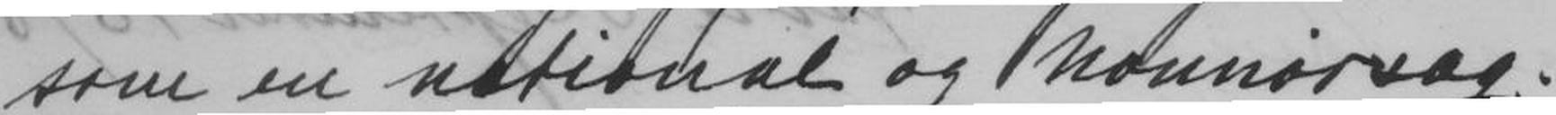som en national og Nonnørsag
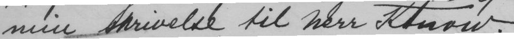min skrivelse til herr Konow.
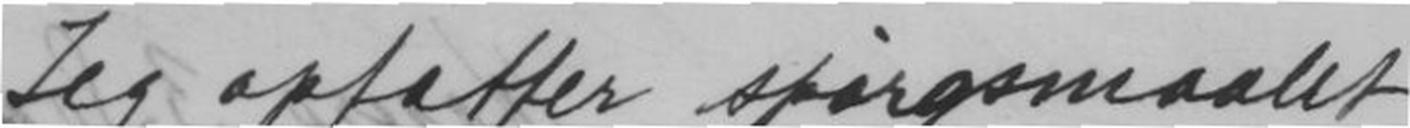Jeg opfatter spørgsmaalet
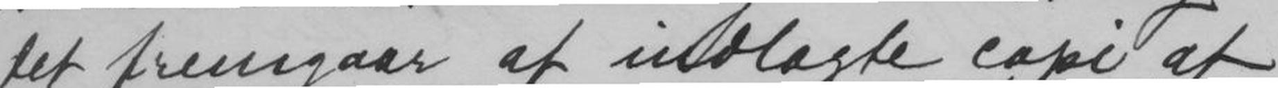det fremgaar af indlagte copi af
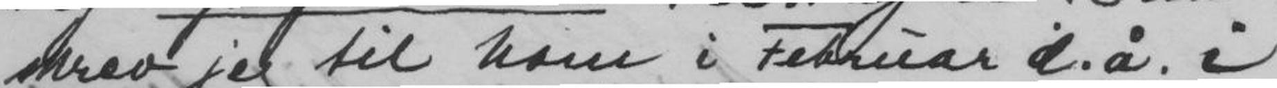skrev jeg til ham i Februar d.å. i
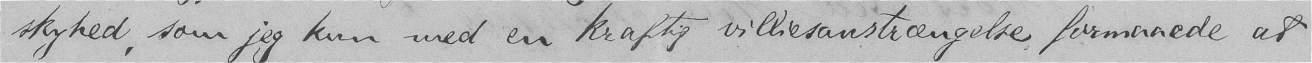skyhed, som jeg kun med en kraftig villiesanstrængelse formaaede at
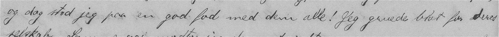og dog stod jeg paa en god fod med dem alle! Jeg gruede blot for Deres
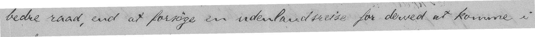bedre raad, end at forsøge en udenlandsreise for derved at komme i
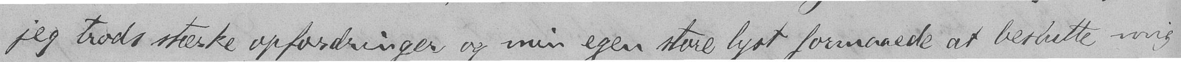jeg trods stærke opfordringer og min egen store lyst formaaede at beslutte mig
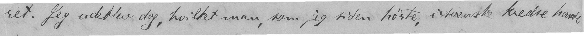ret. Jeg udeblev dog, hvilket man, som jeg siden hørte, i svenske kredse havde
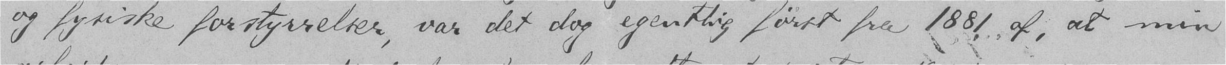og fysiske forstyrrelser, var det dog egentlig først fra 1881 af, at min
